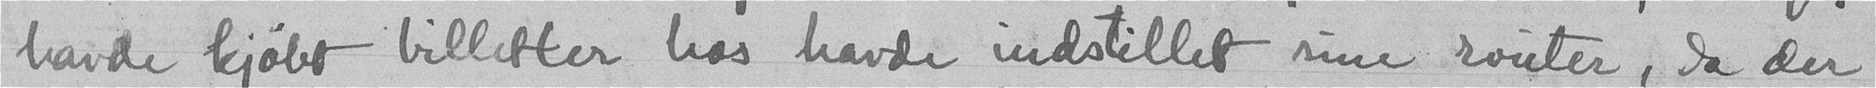havde kjøbt billetter hos havde indstillet sine router, da der
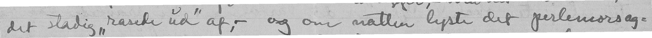det stadig "rasede" ud af, - og om natten lyste det perlemors og-
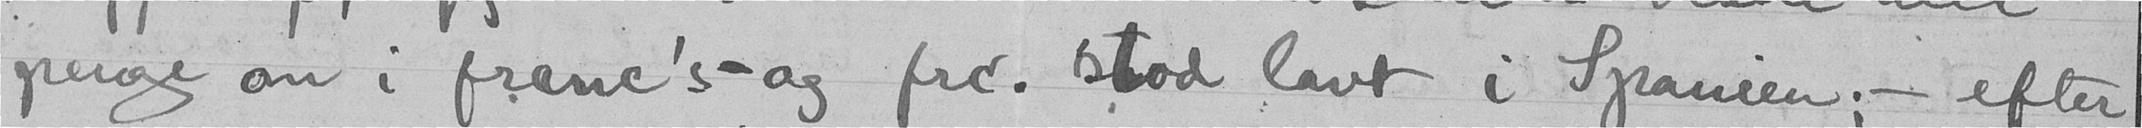penge om i franc's - og frc. stod lavt i Spanien, - efter
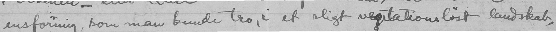ensformig, som man kunde tro, i et sligt vegetationsløst landskab,
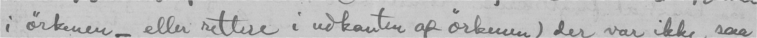i ørkenen, - eller rettere i udkanten af ørkenen) der var ikke saa
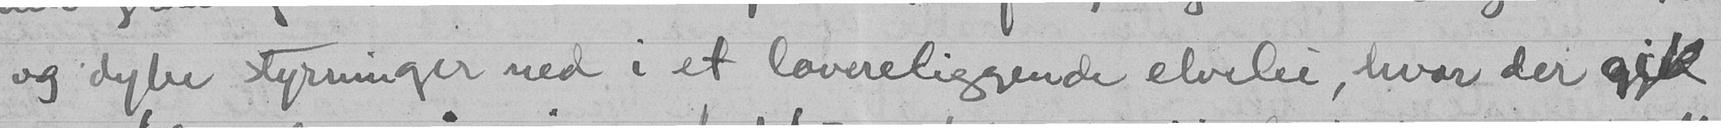og dybe styrninger ned i et lavereliggende elveleie, hvor der gikk
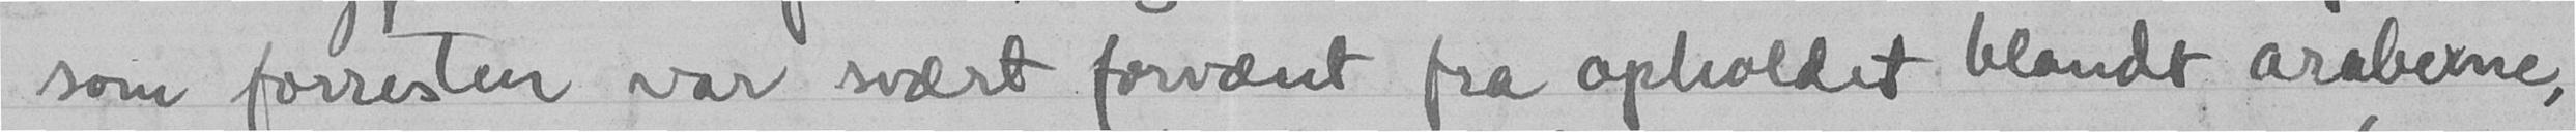som forresten var svært forvænt fra opholdet blandt araberne,
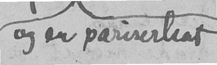og en pariserhat
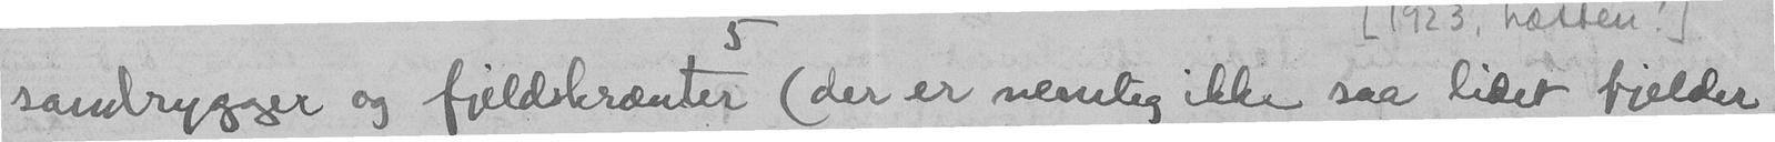sandrygger og fjeldskrænter (der er nemlig ikke saa lidet fielder
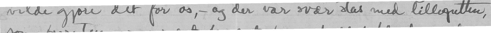vilde gjøre det for os, - og der var svær stas med lillegutten,
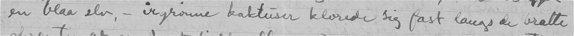en blaa elv, - irgrønne kaktuser klorede sig fast langs de bratte
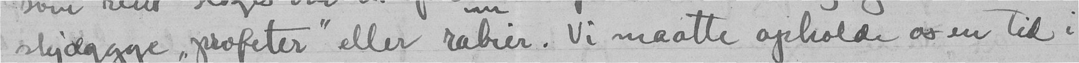skjæggge "profeter" eller rabier. Vi maatte opholde os en tid i
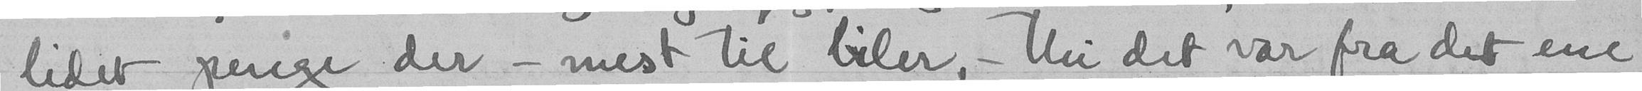lidet penge der - mest til biler, - thi det var fra det ene
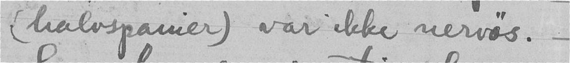(halvspanier) var ikke nervøs. -
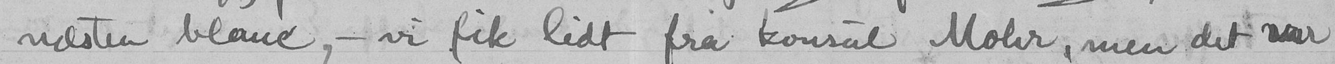næsten blanc,- vi fik lidt fra konsul Mohr, men det var
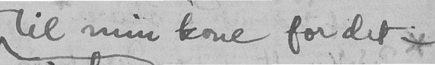til min kone for det -
